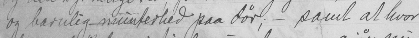og barnlig munterhed paa dør; - samt at hvor
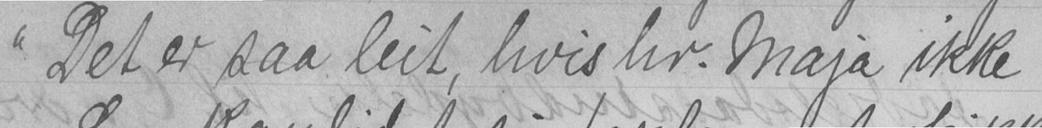"Det er saa leit, hvis hr. Maja ikke
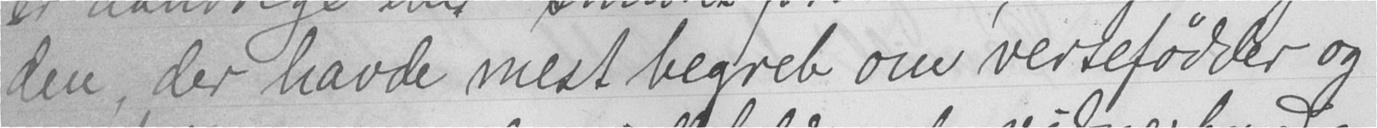den, der havde mest begreb om versefødder og
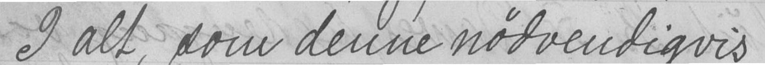I alt, som denne nødvendigvis
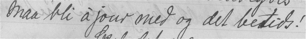maa bli à jour med og det betids!
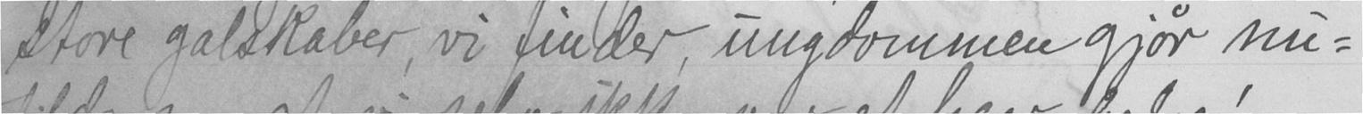store galskaber, vi finder, ungdommen gjør nu-
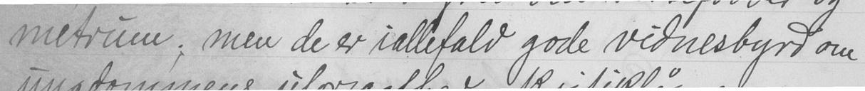metrum; men de er iallefald gode vidnesbyrd om
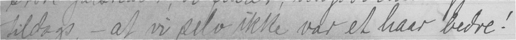tildags - at vi selv ikke var et haar bedre!
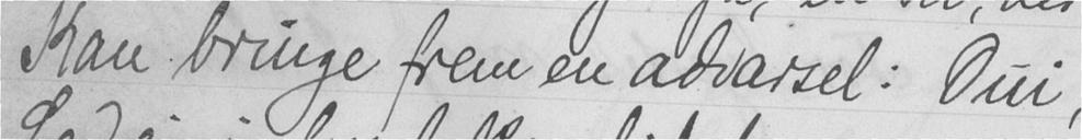Kan bringe frem en advarsel: Oui,
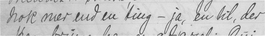nok mer end en ting - ja, en til, der
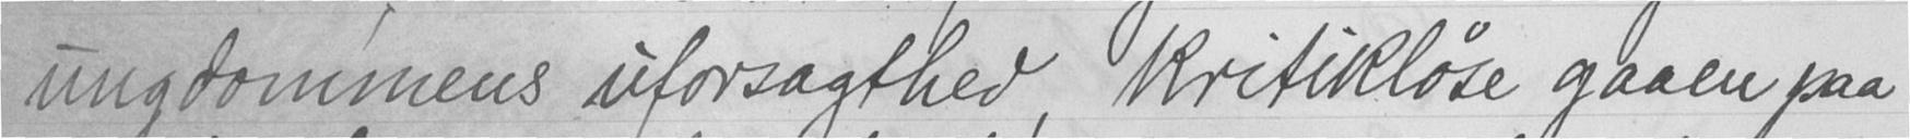ungdommens uforsagthed, kritikløse gaaen paa
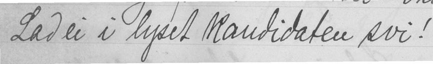Lad ei i lyset kandidaten svi!
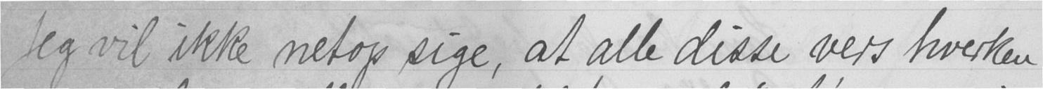Jeg vil ikke netop sige, at alle disse vers hverken
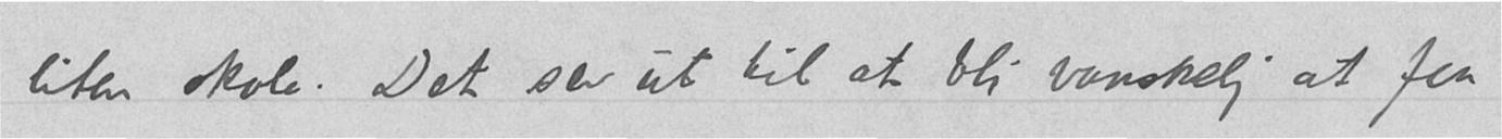liten skole. Det ser ut til at bli vanskelig at faa
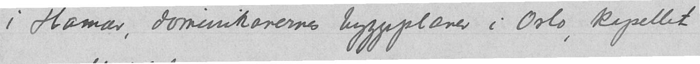i Hamar, dominikanernes byggeplaner i Oslo, kapellet
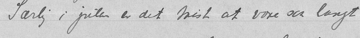Særlig i julen er det trist at være saa langt
